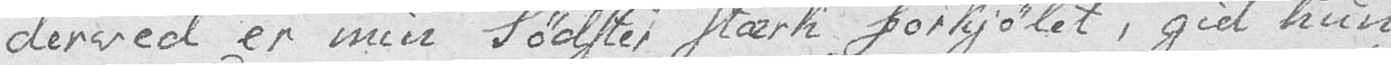derved er min Sødster stærk forkjølet, gid hun
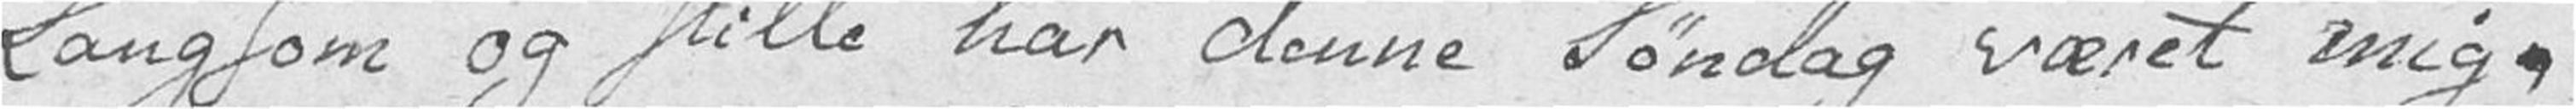Langsom og stille har denne Søndag været mig.
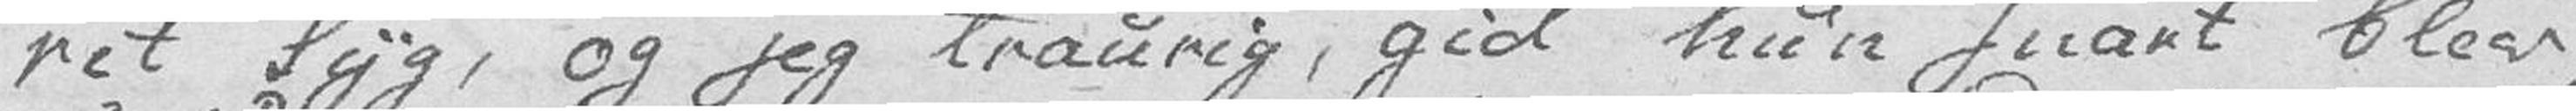ret Syg, og jeg traurig, gid hun snart blev
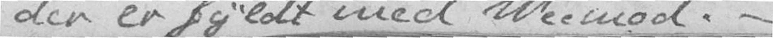der er fyldt med Veemod. –
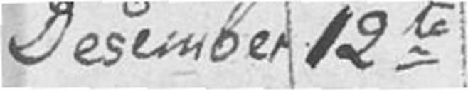Desember 12te
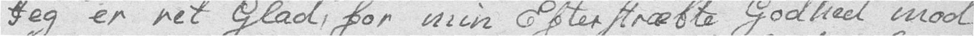Jeg er ret Glad, for min Efterstræbte Godhed mod
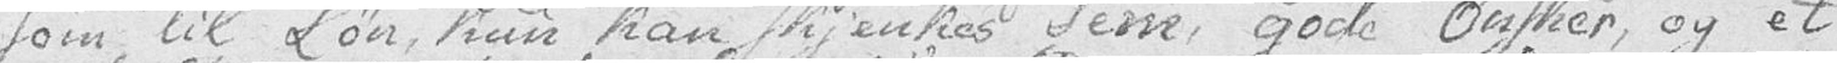som lik Løn, kun kan skjenkes Dem, gode Ønsker, og et
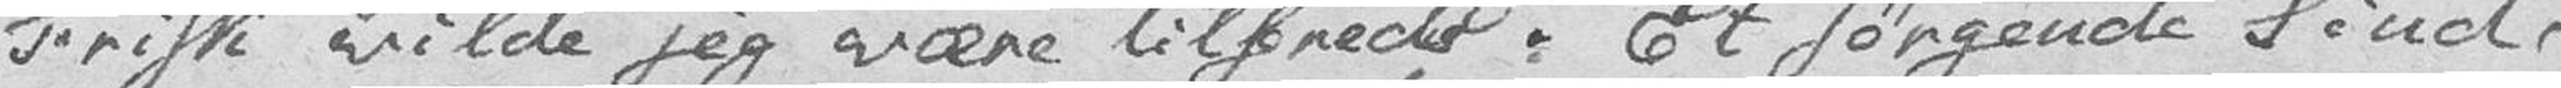Frisk vilde jeg være tilfreds. Et sørgende Sind,
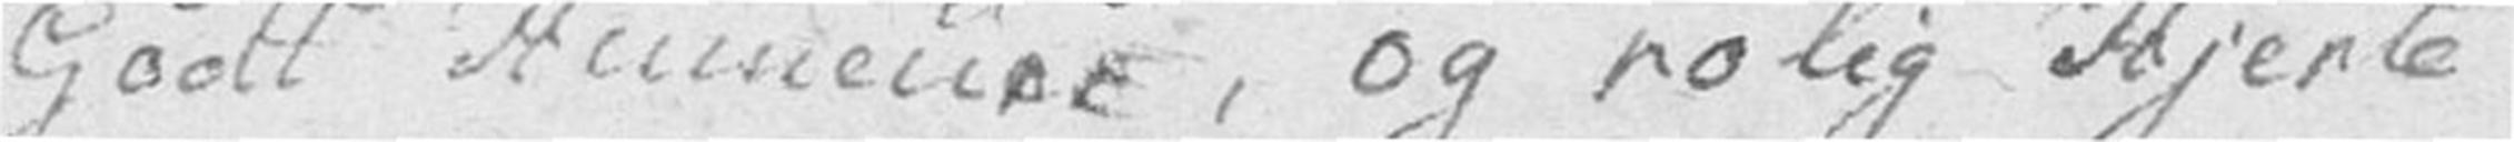Godt Humeur, og rolig Hjerte
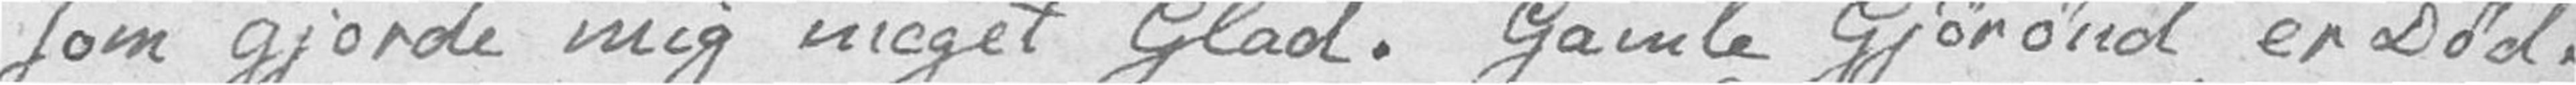som gjorde mig meget Glad. Gamle Gjørønd er Død.
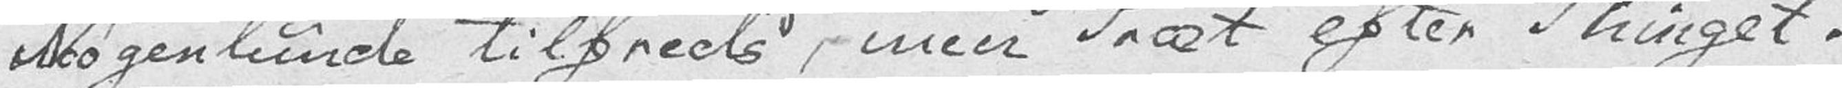Nogenlunde tilfreds, men træt efter Thinget.
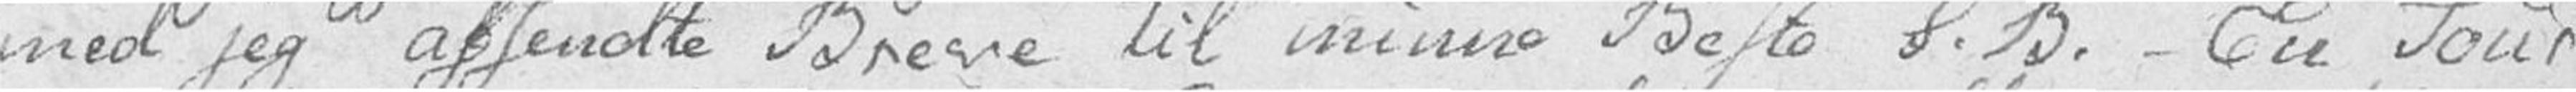med jeg afsendte Breve til minne Beste S. B. – En Tour
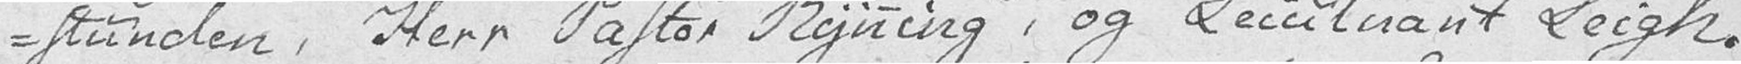-stunden, Herr Pastor Rynning, og Leiutnant Leigh.
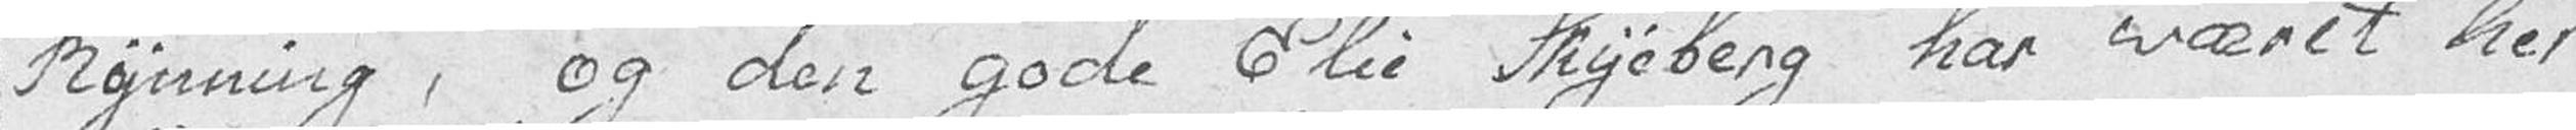Rynning, og den gode Elie Skyeberg har været her
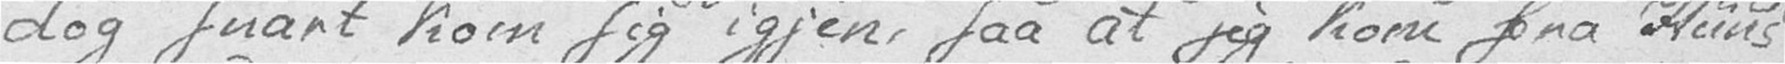dog snart kom sig igjen, saa at jeg kom fra Huus
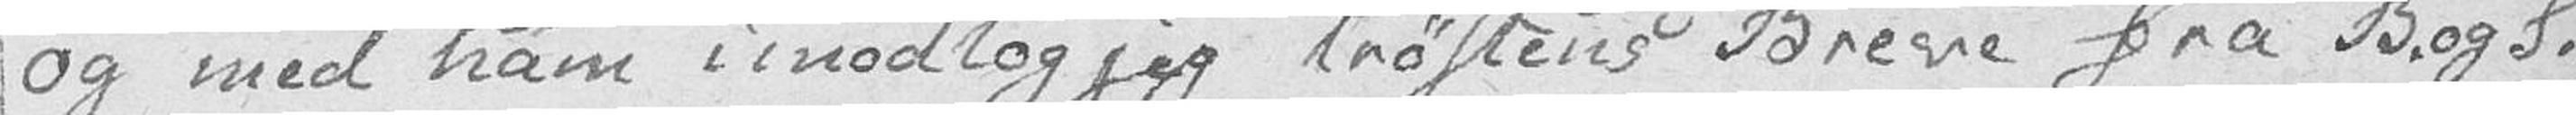og med ham imodtog jeg trøstens Breve fra B. og S.
